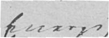Energi
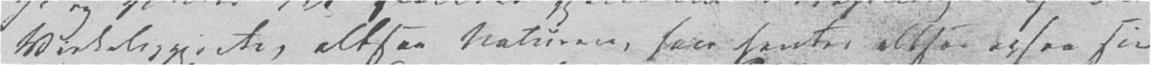Virkeliggjorte, altsaa Naturen, han henter altsaa ogsaa sin
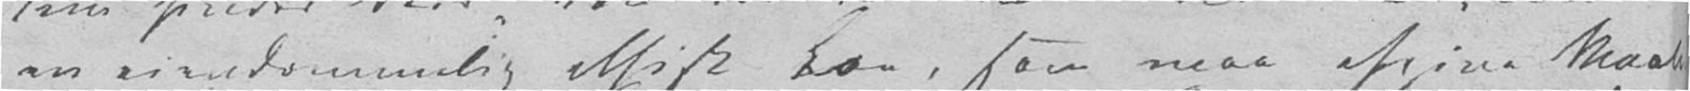en eiendommelig ethisk Lov, som maa afgive Maale-
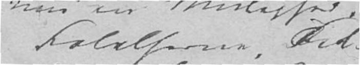Følelserne, Til-
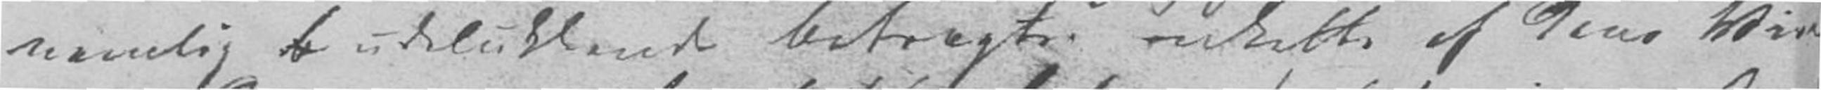nemlig udelukkende betragter enkelte af dens Virk-
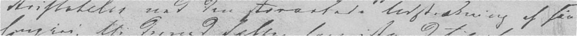Aristoteles ved den storartede Udstrækning af sin
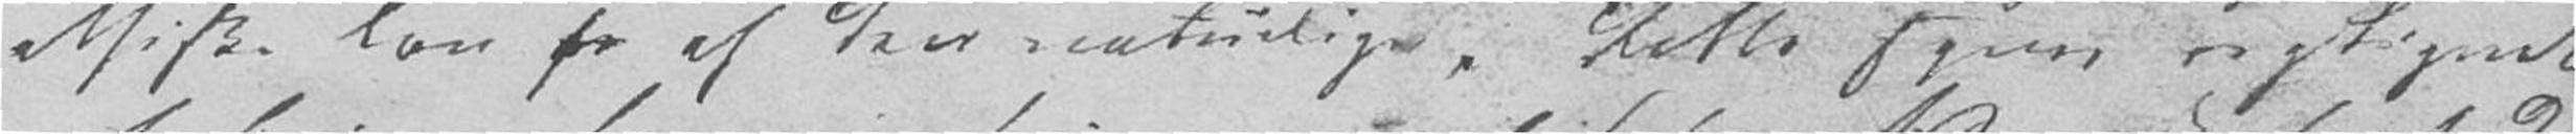ethiske Lov fr af den naturlige. Dette synes rigtignok
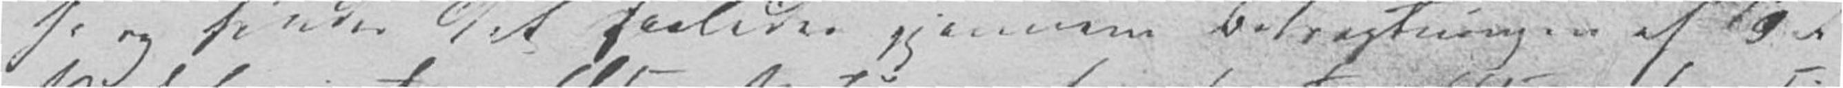se og finder det saaledes gjennem Betragtningen af det
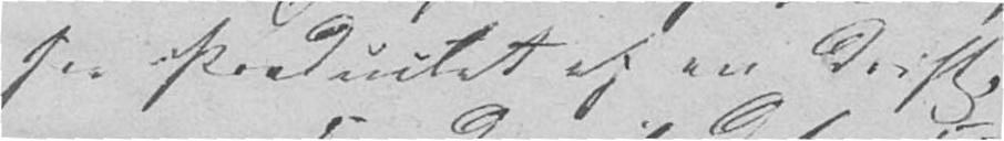saa Productet af en Drift.
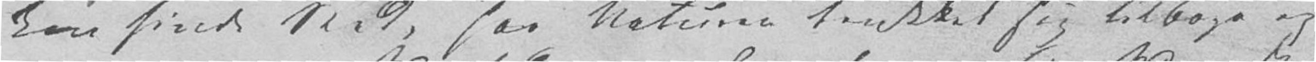kan finde Sted, har Naturen trukket sig tilbage og
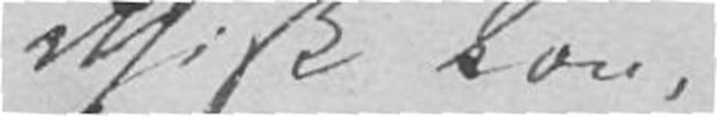ethisk Lov,
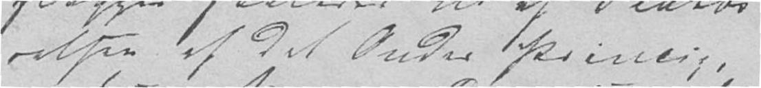relsen af det Ondes Princip.
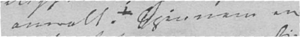overalt . Gjennem en
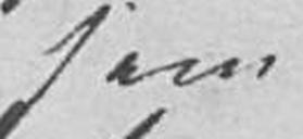som
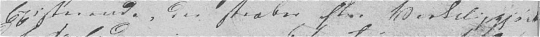Existerende, der stræber efter Virkeliggjørel-
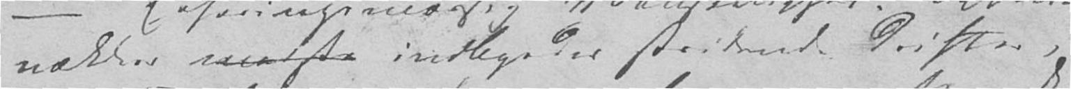vækker maaske indbyrdes stridende Drifter,
